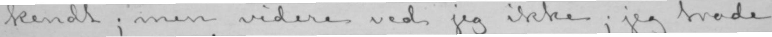kendt; men videre ved jeg ikke; jeg trode
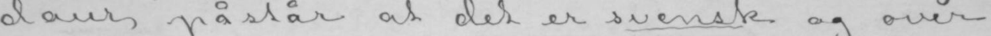daur påstår at det er svensk og over-
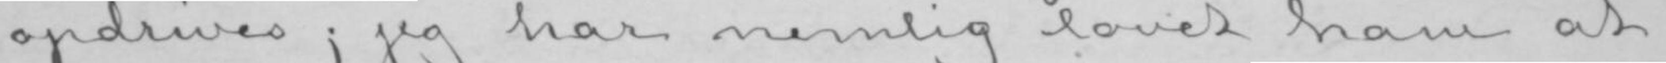opdrives; jeg har nemlig lovet ham at
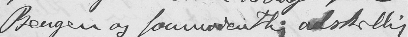Bergen og formodentlig adskillig
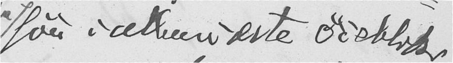før i allersidste øieblik,
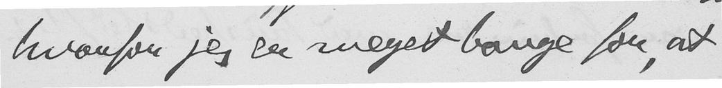hvorfor jeg er meget bange for, at
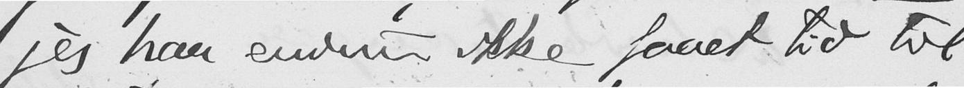jeg har endnu ikke faaet tid til
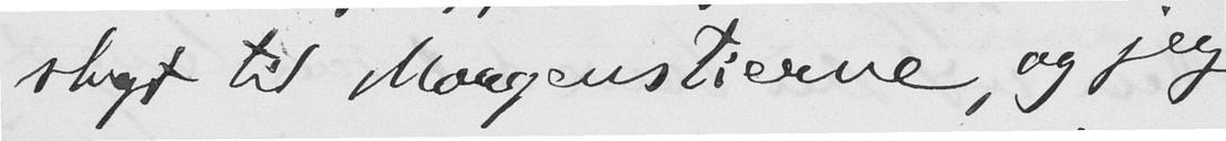sligt til Morgenstierne, og jeg
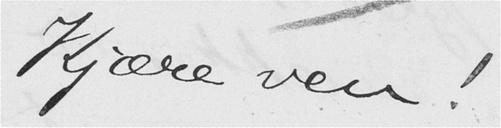Kjære ven!
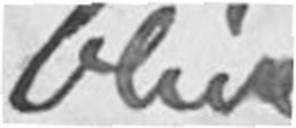blive
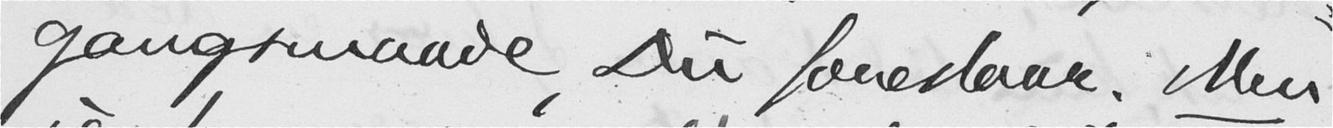gangsmaade, Du foreslaar. Men
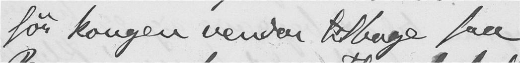før kongen vender tilbage fra
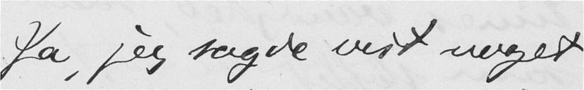Ja, jeg sagde vist noget
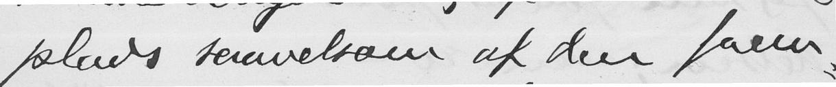plads saavelsom af den frem-
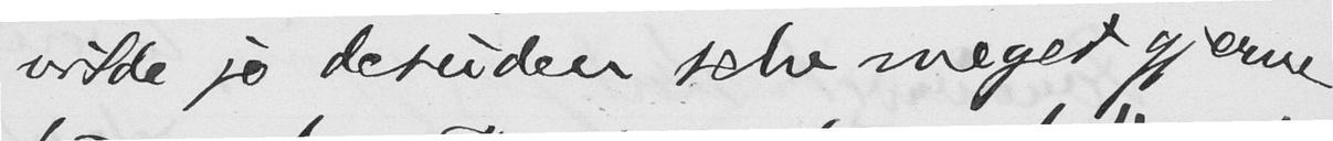vilde jo desuden selv meget gjerne
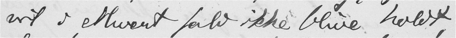vil i ethvert fald ikke blive holdt,
